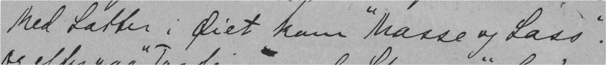Med Latter i Øiet kom "Masse og Lass".
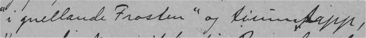i gnellande Frosten" og linumslapp,
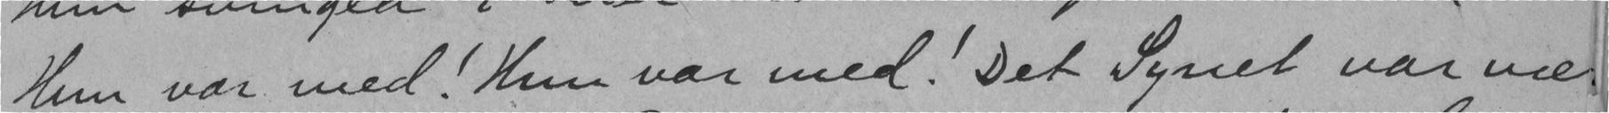Hun var med! Hun var med! Det Synet var nær
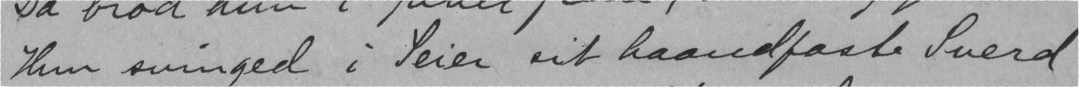Hun svinged i Seier sit haandfaste Sverd
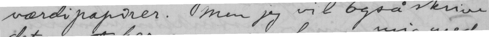værdipapirer. Men jeg vil også skrive
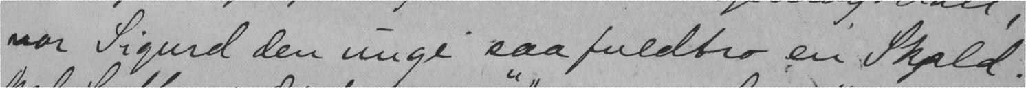var Sigurd den unge saafuldtro en Skald.
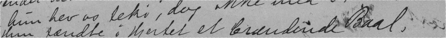Hun tændte i Hjertet et brændende Baal;
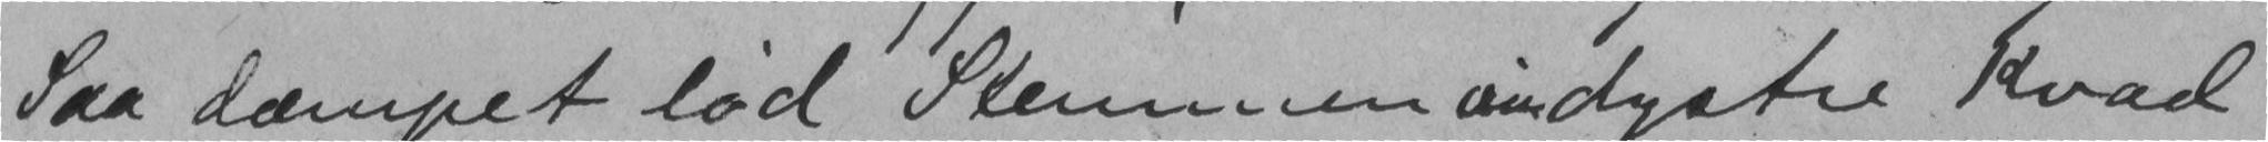Saa dæmpet lød Stemmen sin dystre kvad
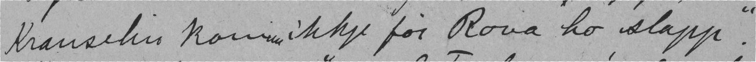Kranselin kom "ikkje før Rova ho slapp".
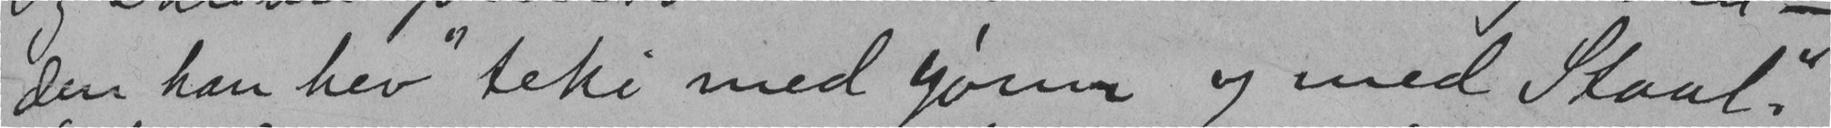den han hev "teki med søm og med Staal".
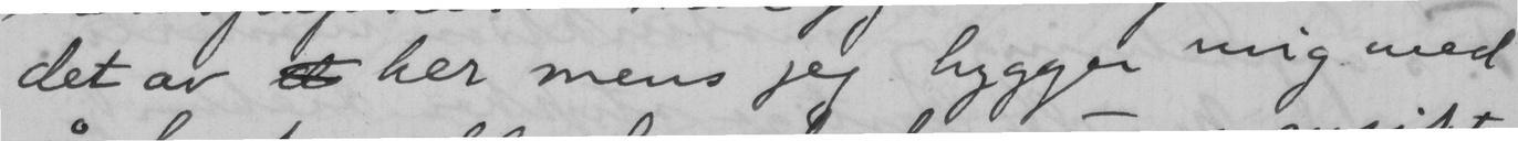det av  her mens jeg hygger mig med
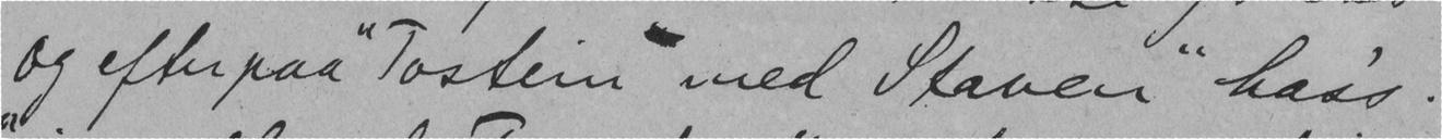og efterpaa "Torstein med Staven" hass.
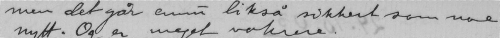men det går ennu likså sikkert som noe
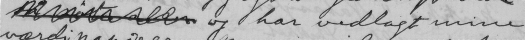og har vedlagt mine
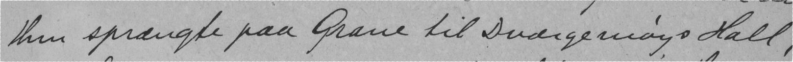Hun sprængte paa Grane til Dværgemøyjs Hall,
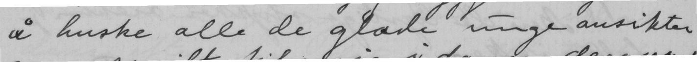å huske alle de glade unge ansikter
